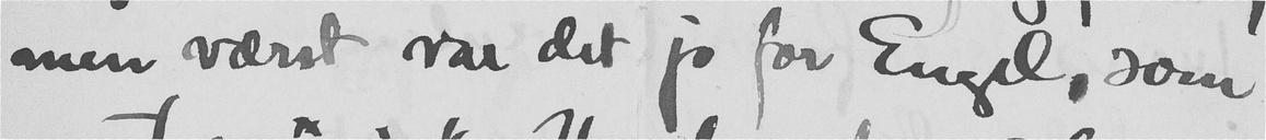men værst var det jo for Engel, som
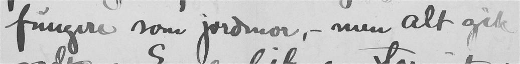fungere som jordmor, - men alt gik
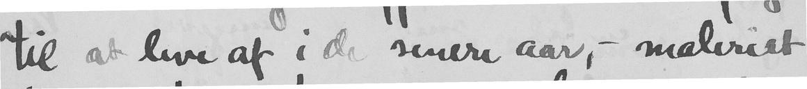til at leve af i de senere aar, - maleriet
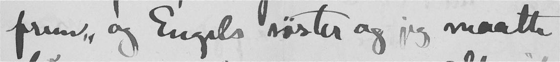frem, og Engels søster og jeg maatte
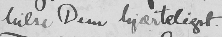hilse Dem hjærteligst.
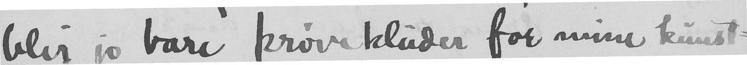blir jo bare prøvekluder for mine kunst-
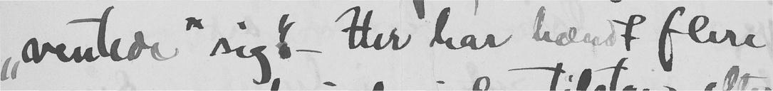"ventede sig". - Her har hændt flere
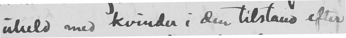uheld med kvinder i den tilstand efter
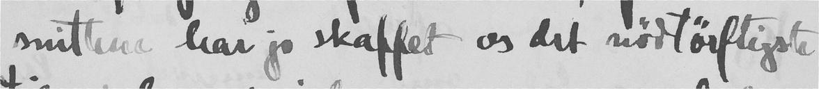snittene har jo skaffet os det nødtørftigste
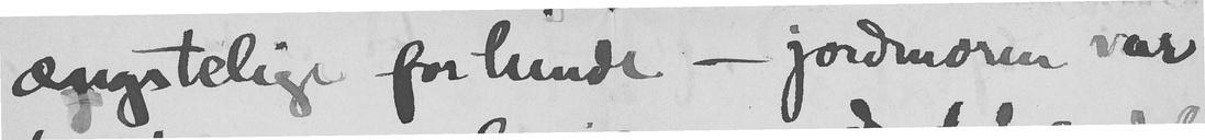ængstelige for hende - jordmoren var
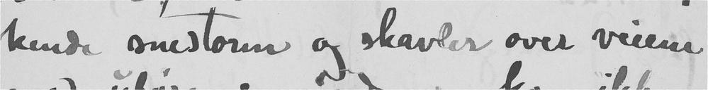kende snestorm og skavler over veiene
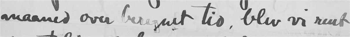maaned over beregnet tid, blev vi rent
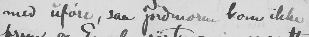med uføre, saa jordmoren kom ikke
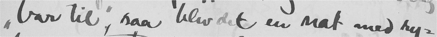"bar til", saa blev det en nat med ry-
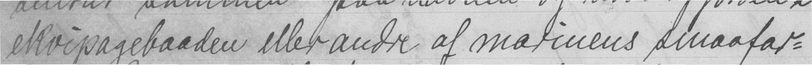ekvipagebaaden eller andre af marinens smaafar-
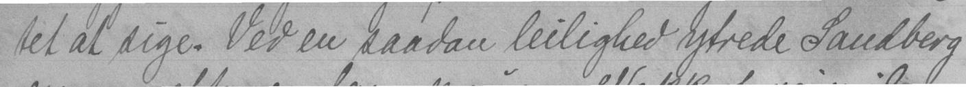tet at sige. Ved en saadan leilighed ytrede Sandberg
